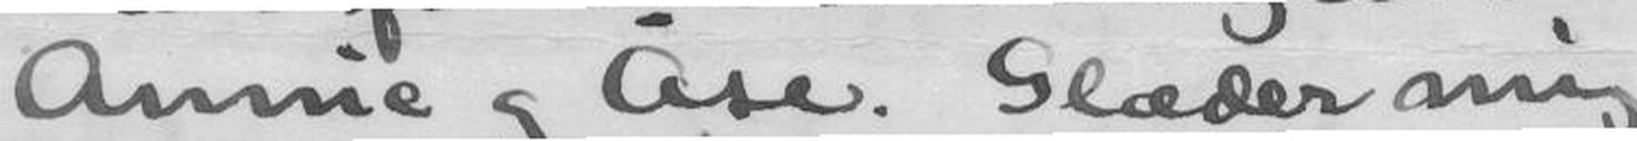Annie og Åse. Glæder mig
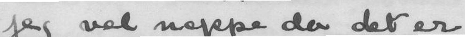jeg ved næppe da det er
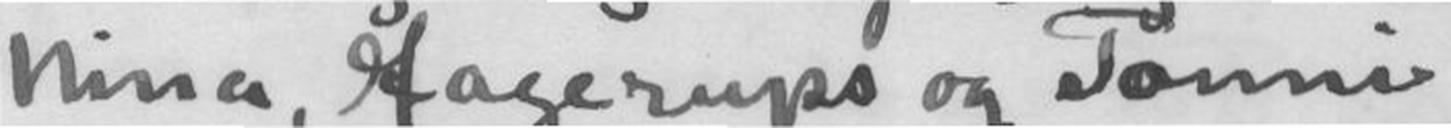Nina, Hagerups og Tonni.
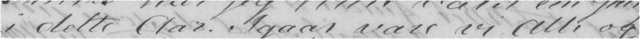i dette Aar. Igaar vare vi Alle og
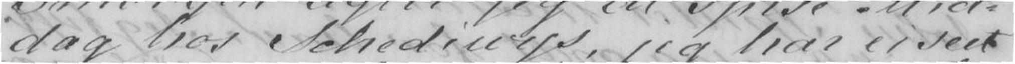dag hos Schediwys, jeg har ei seet
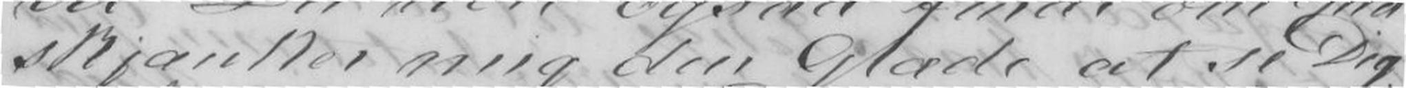skjænker mig den Glæde at se Dig
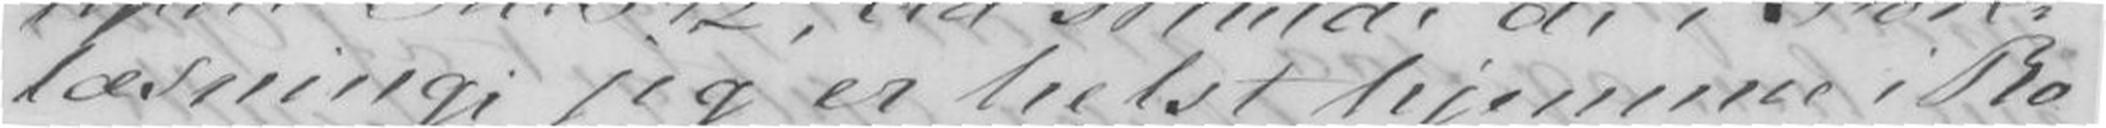læsninge; jeg er helst hjemme i Ro
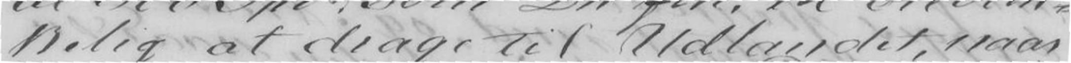kelig at drage til Udlandet, naar
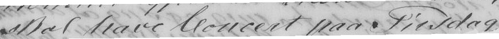skal have Concert paa Tirsdag
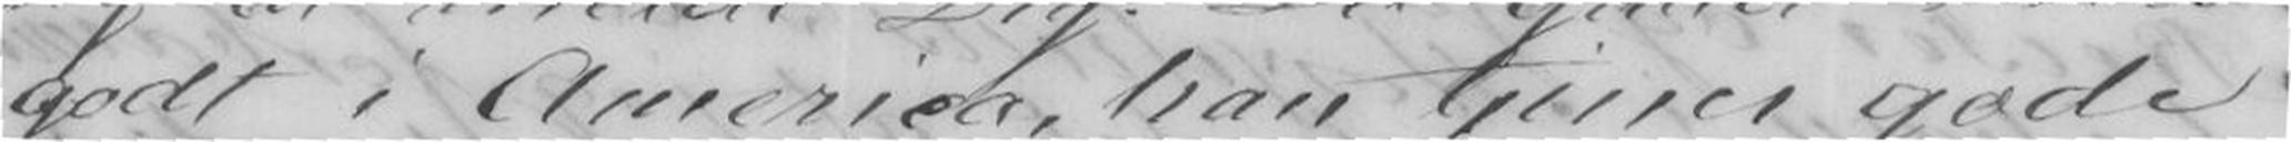godt i America, han tjener gode
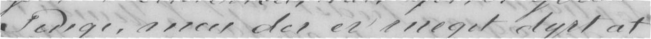Penge, men der er meget dyrt at
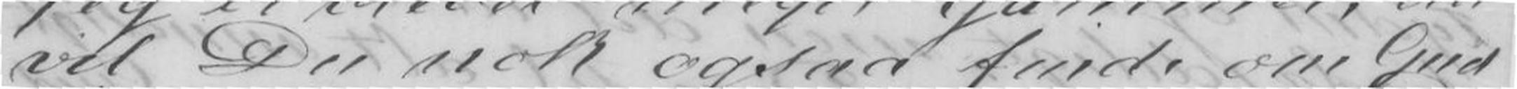vil Du nok ogsaa finde om Gud
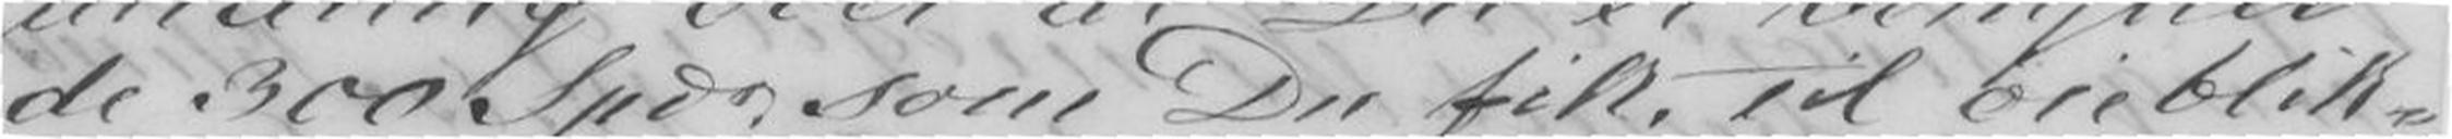de 300 Spdo, som Du fik, til øieblik-
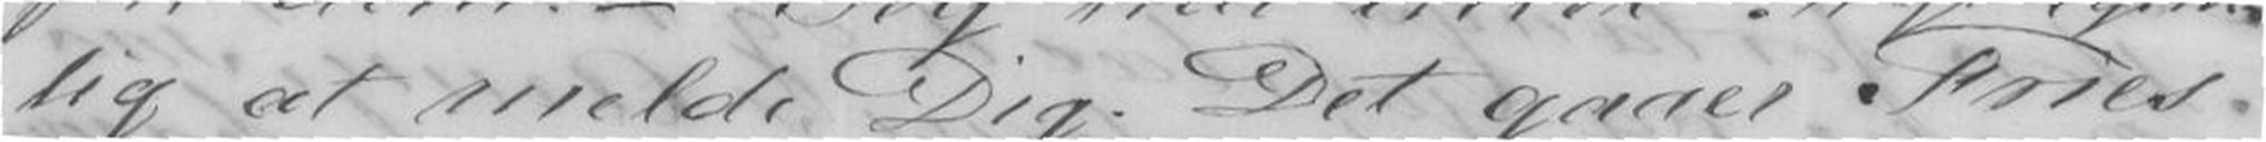lig at melde Dig. Det gaaer Fries
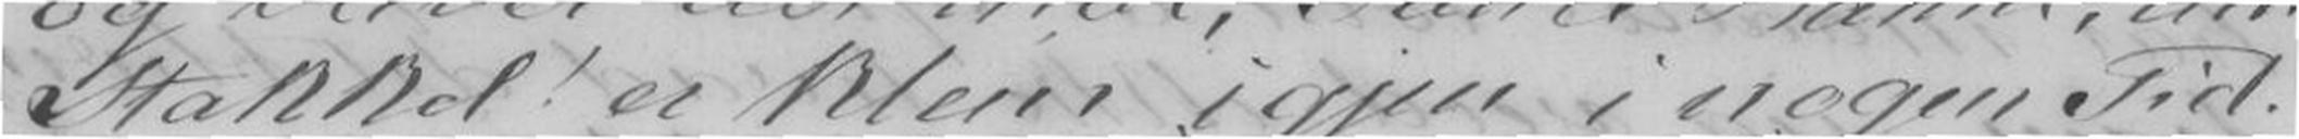Stakkel! er klein igjen i nogen Tid.
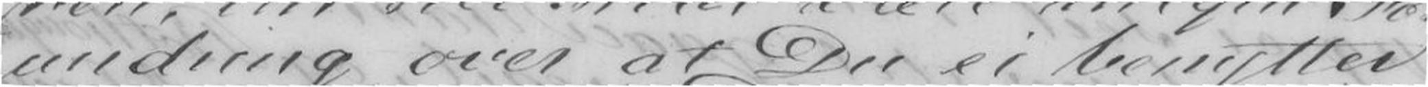undring over at Du ei benytter
